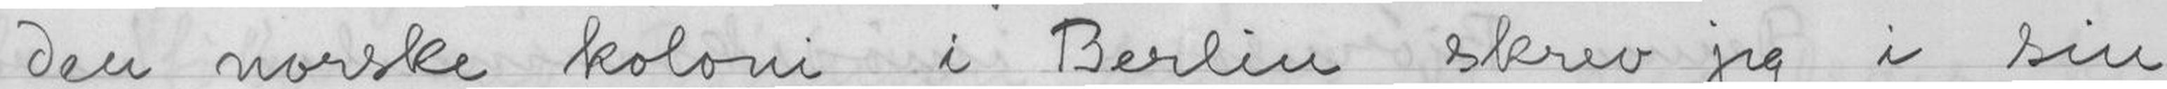den norske koloni i Berlin skrev jeg i sin
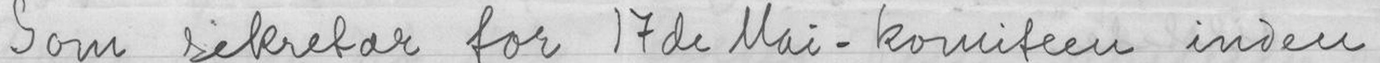Som sekretær for 17de Mai-komiteen inden
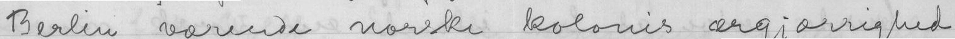Berlin værende norske kolonis ærgjærrighed
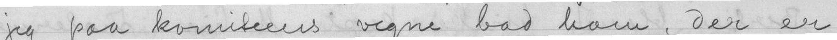jeg paa komiteens vegne bad ham, der er
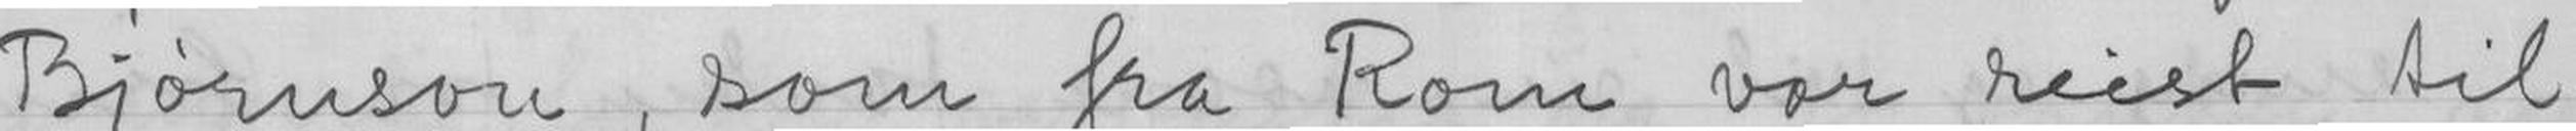Bjørnson, som fra Rom var reist til
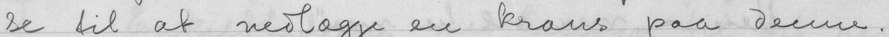se til at nedlægge en krans paa denne.
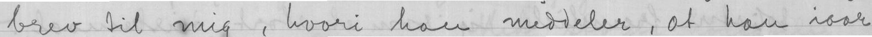brev til mig, hvori han meddeler, at han iaar
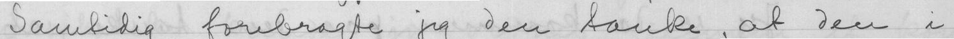Samtidig forebragte jeg den tanke, at den i
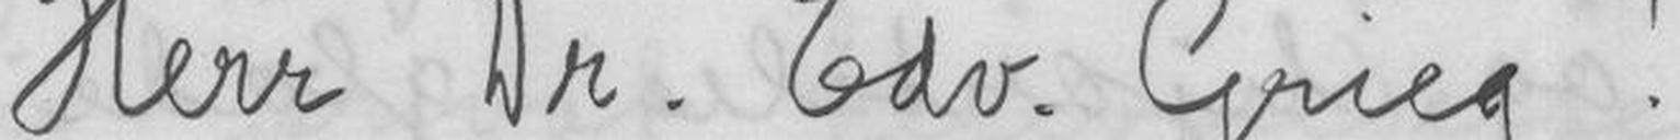Herr Dr. Edv. Grieg!
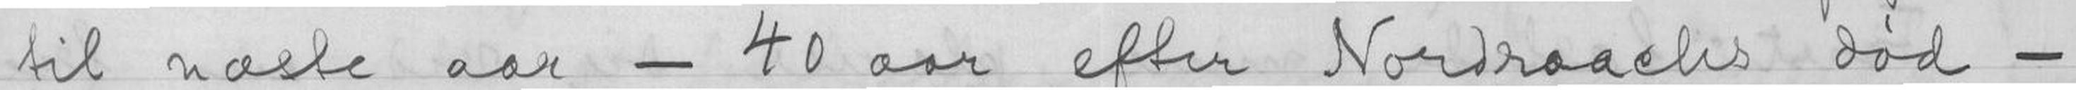til næste aar - 40 aar efter Nordraachs død -
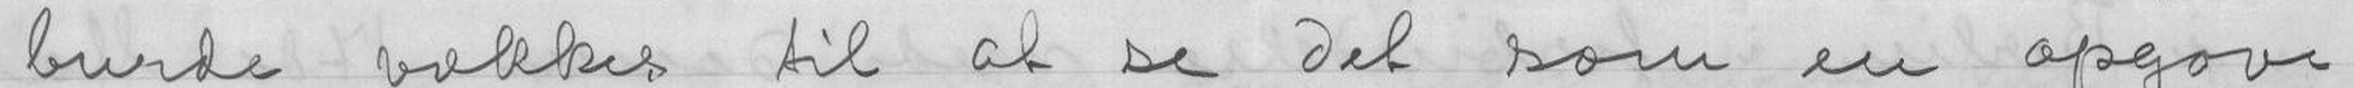burde vækkes til at se det som en opgave
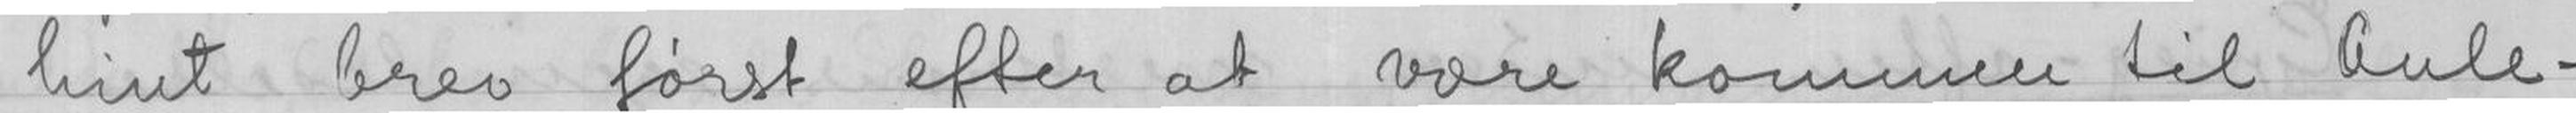hint brev først efter at være kommen til Aule-
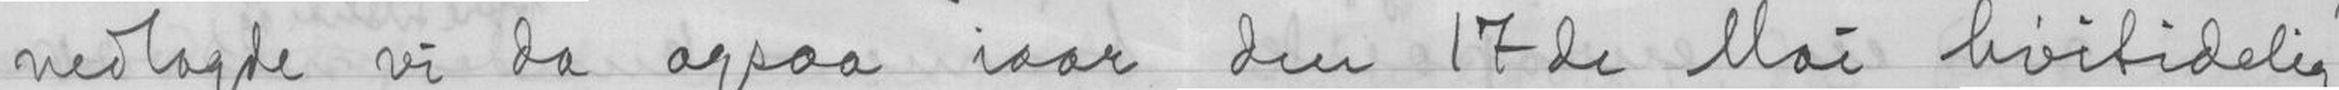nedlagde vi da ogsaa iaar den 17de Mai høitidelig
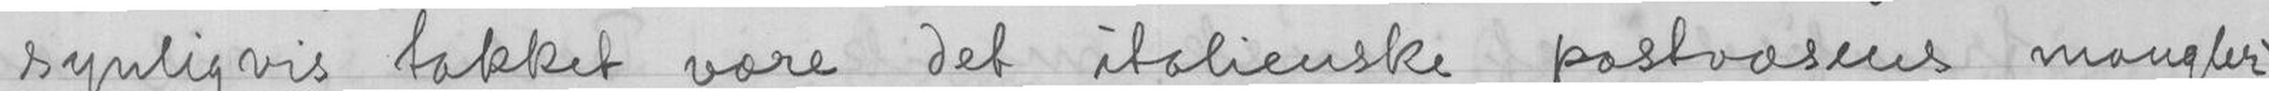synligvis takket være det italienske postvæsens mangler
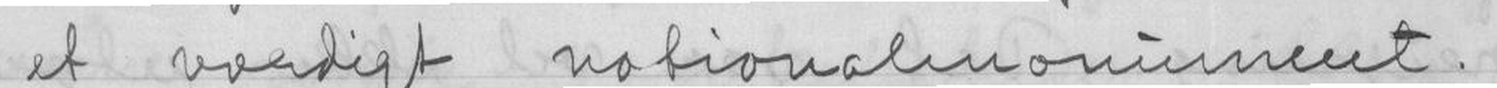et værdigt nationalmonument.
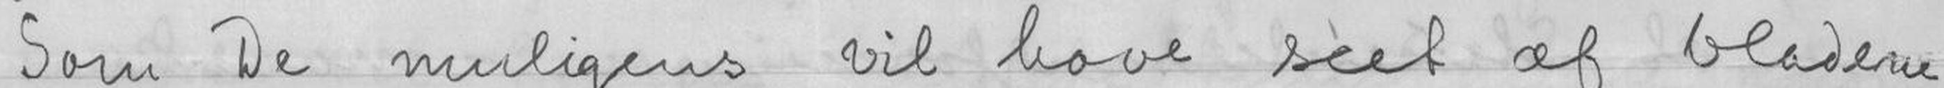Som De muligens vil have séet af bladene
